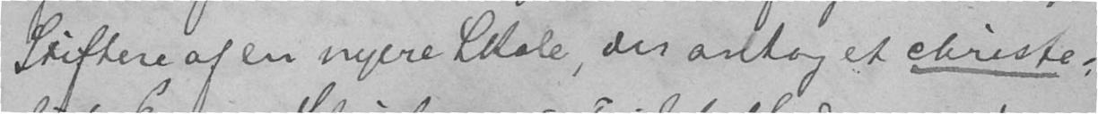Stiftere af en nyere Skole der antog et christe-
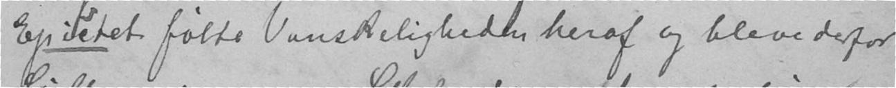Epictet følte Vanskeligheden heraf og bleve derfor
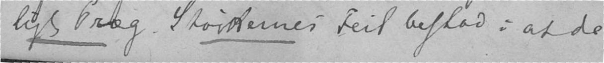ligt Præg. Stoikernes Feil bestod i at de
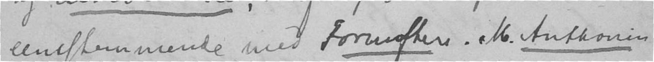eensstemmende med Fornuften. M. Antonien
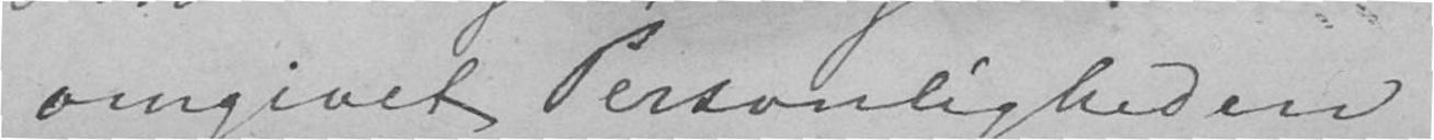omgivet Personligheden,
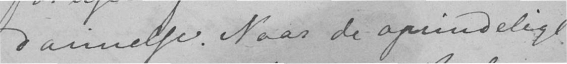dannelse. Naar de oprindelige
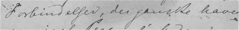Forbindelser, der ganske have
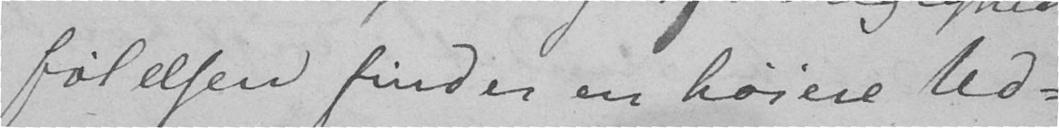følelsen finder en høiere Ud-
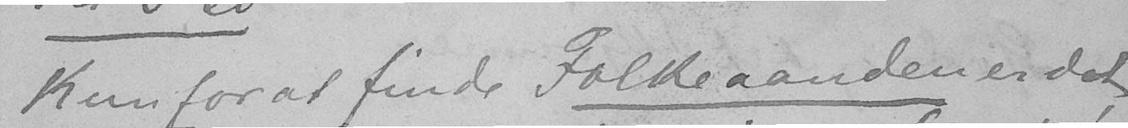Kun for at finde Folkeaanden er det,
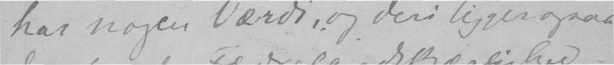har nogen Værdi, og deri ligger ogsaa
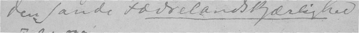den sande Fædrelandskjærlighed
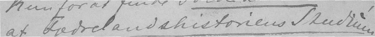at Fædrelandshistoriens Studium
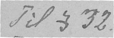Til § 32.
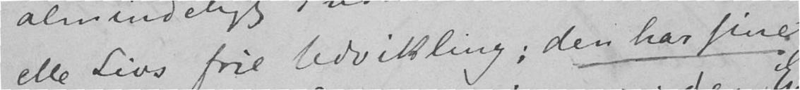elle Livs frie Udvikling; den har sine
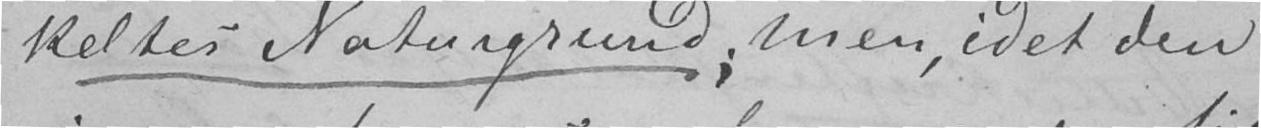keltes Naturgrund; men, idet den
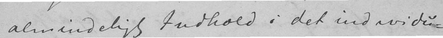almindeligt Indhold i det individu-
